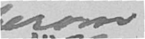herom
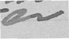er
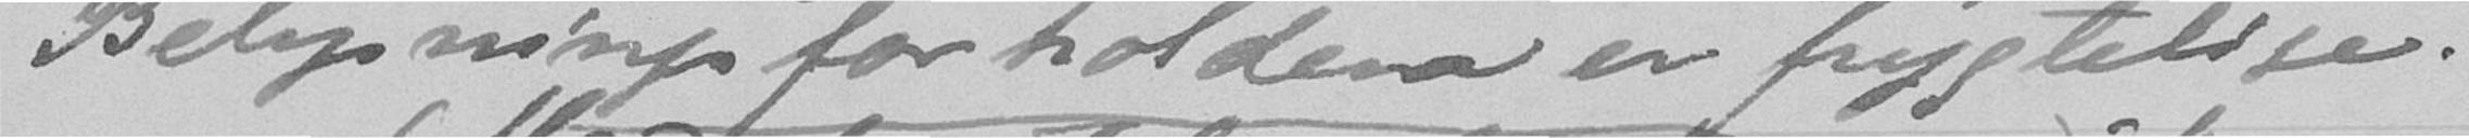Belysningsforholdene er frygtelige.
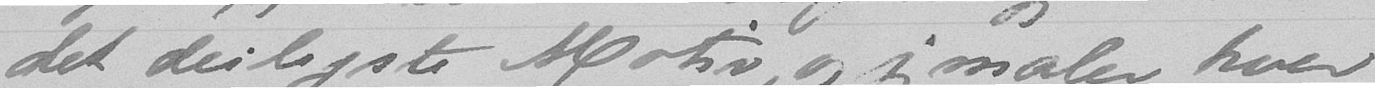det deiligste Motiv, og jeg maler hver
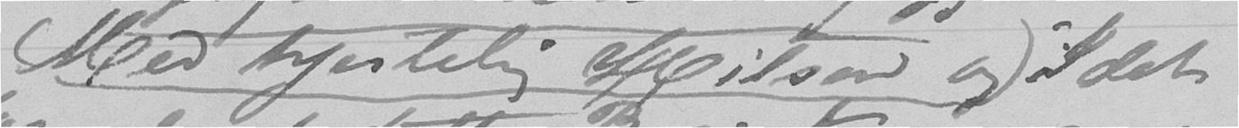Med hjertelig Hilsen og i det
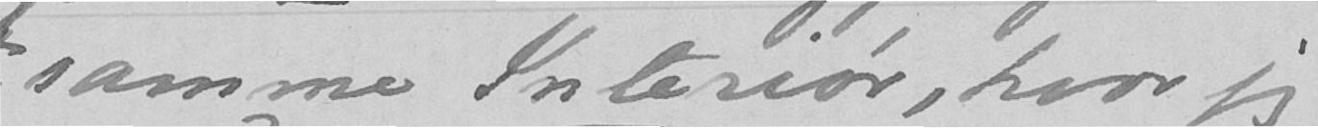samme Interiør, hvor jeg
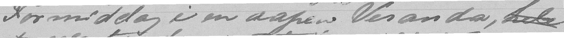Formiddag i en aapen Veranda, hele
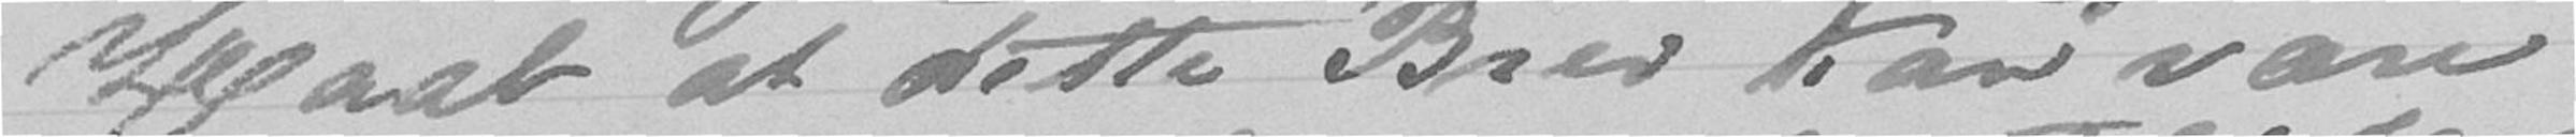Haab at dette Brev kan være
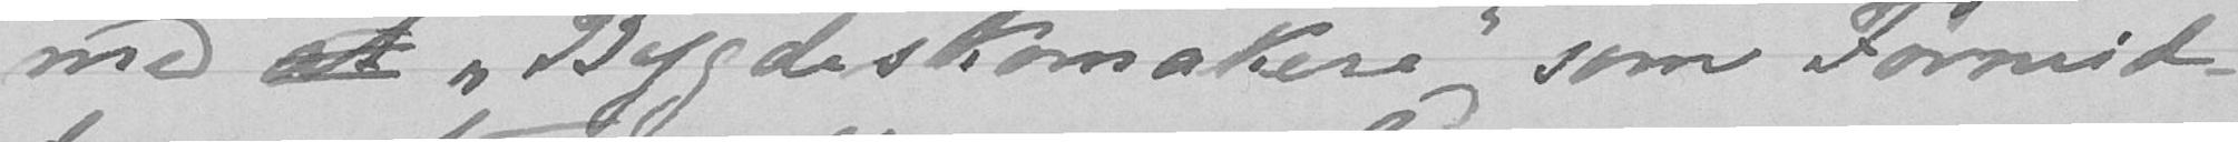med at "Bygdeskomakere" som Formid-
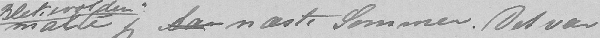malte jeg Aar næste Sommer. Det var
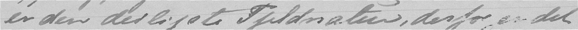er den deiligste Fjeldnatur, derfor er det
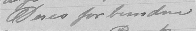Deres forbundne
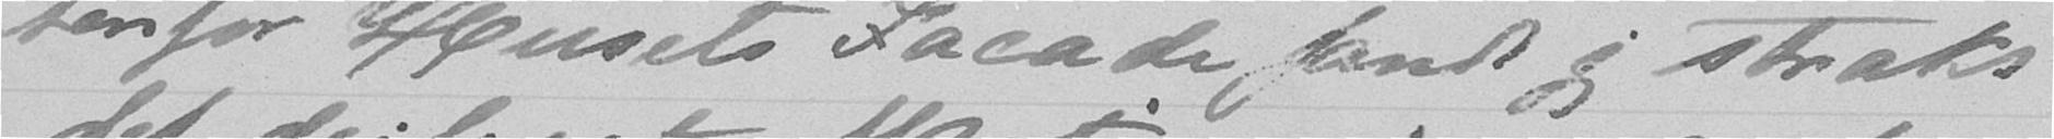tenfor Husets Facade fandt jeg straks
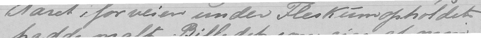Aaret i forveien under Fleskumopholdet
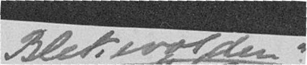Blekevolden
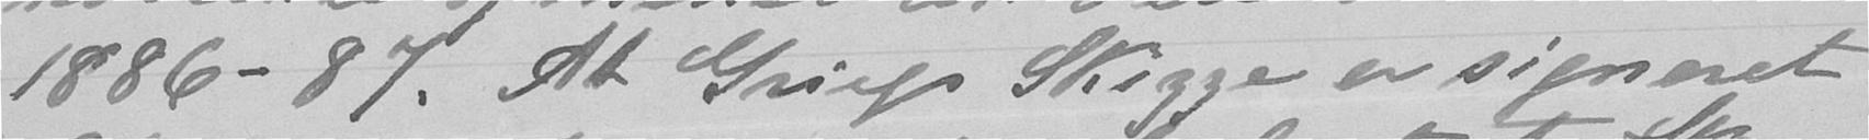1886-87. At Griegs Skizze er signeret
